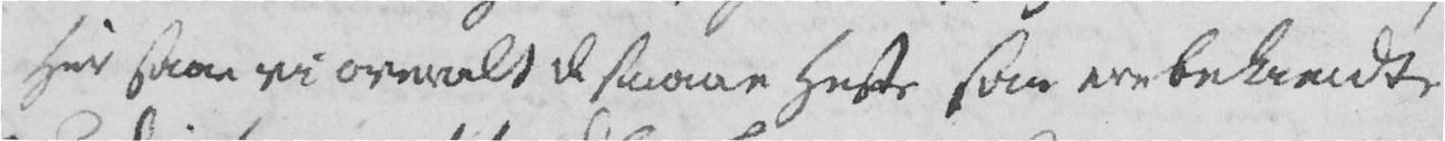Her saa vi overalt de smaae Heste som ere bekiendte
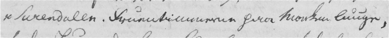i Surendalen. Fruentimmerne paa Marken luuge,
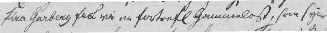Paa Garberg fik vi en fortrefl Gammelost, som synes
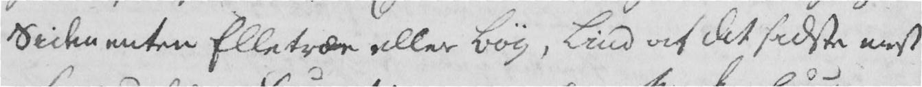Siden enten Elletræe eller Bøg, Lind af det sidste mest
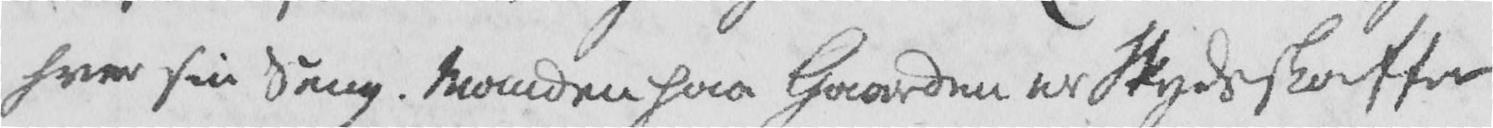hver sin Seng. Manden paa Gaarden er Skydsskaffer
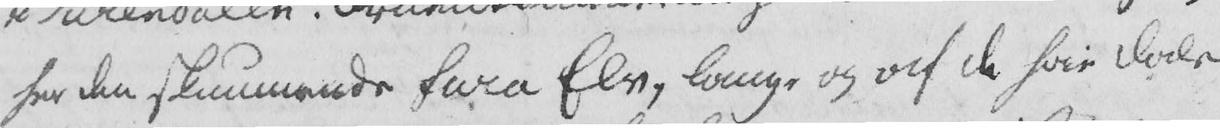her den skummende Sura Elv, lange og af de høie Dale
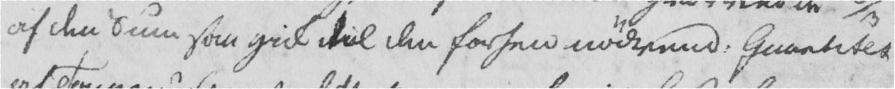af den Sum som gik til den forhen nødvend. Quantitet
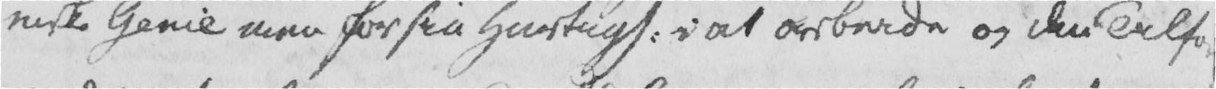niske Genie men for sin Hurtigh. i at arbeide og den Tilfo-
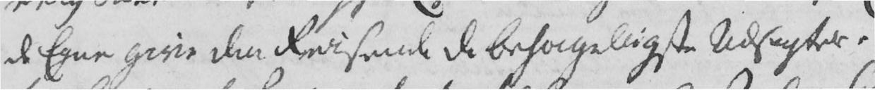de Egne give den Reisende de behageligste Udsigter.
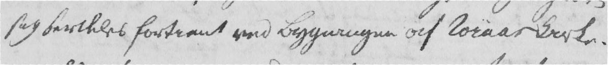sig serdeles fortient ved Bygningen af Røraas Kirke.
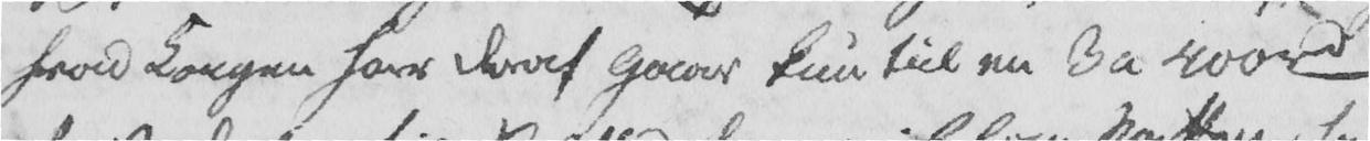hvad Kongen har deraf gaar kun til en 3 a 400 rd -
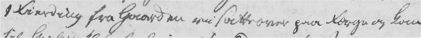1 Fierding fra Gaarden vi satte over paa Færge og kom
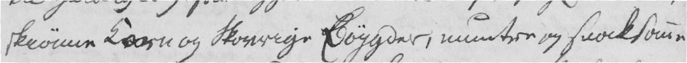skiønne Korn og Skovrige Bøygder, muntre og snaksome
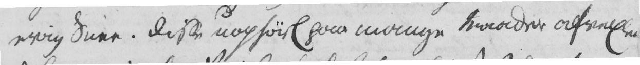evig Snee. Disse uophørl paa mange Maader afvexlen-
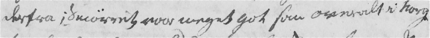derfra; Smørret var meget got som overalt i Norge
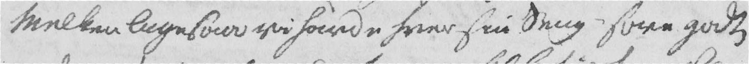Melken ligesaa vi havde hver sin seng - sove godt
